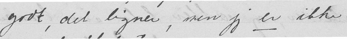godt, det ligner, men jeg er ikke
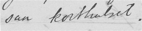saa korthalset.
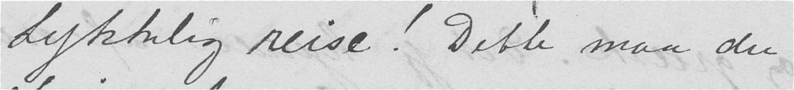Lykkelig reise! Dette maa du
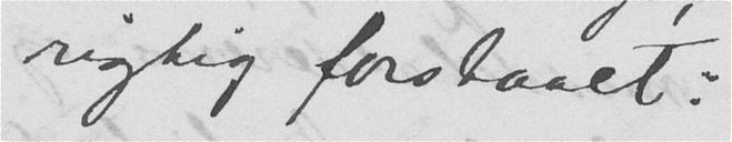rigtig forstaaet:
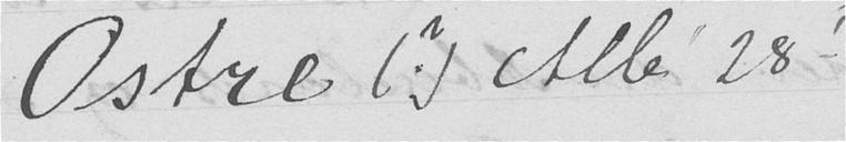Østre (?) Allé 28 I
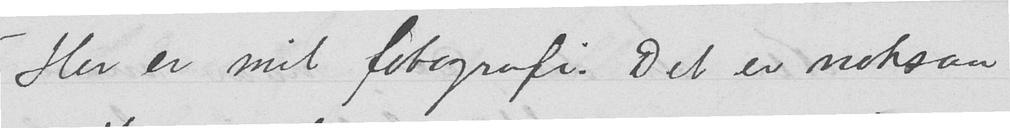Her er mit fotografi. Det er noksaa
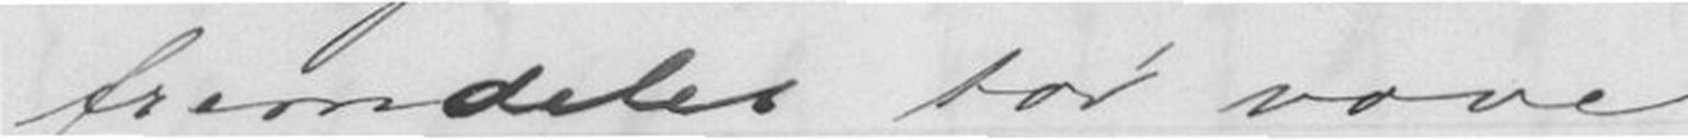fremdeles tør vove
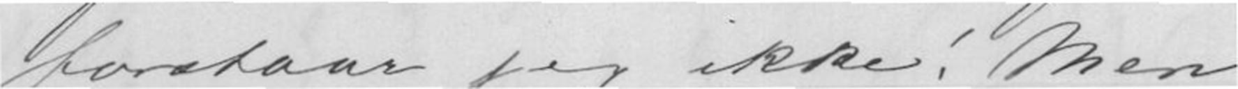forstaar jeg ikke! Men
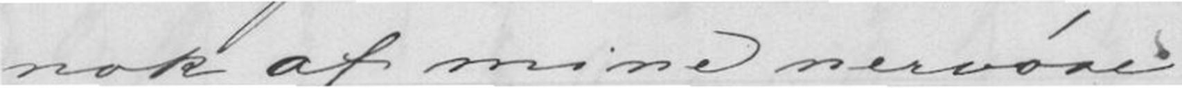nok af mine nervøse
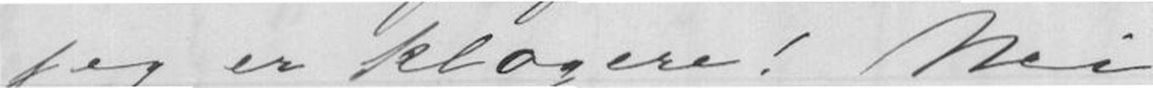jeg er klogere! Nei
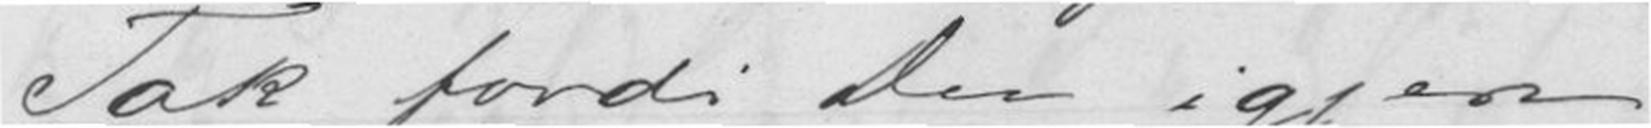Tak fordi Du igjen
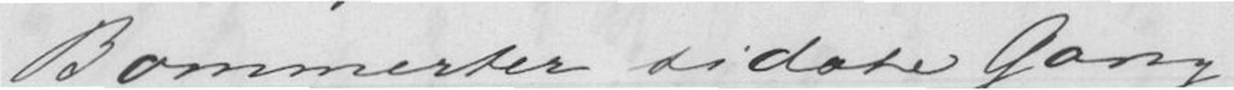bommerter sidste Gang
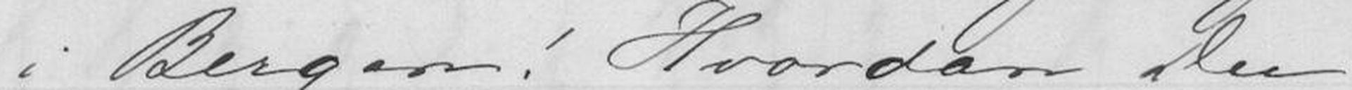i Bergen! Hvordan Du
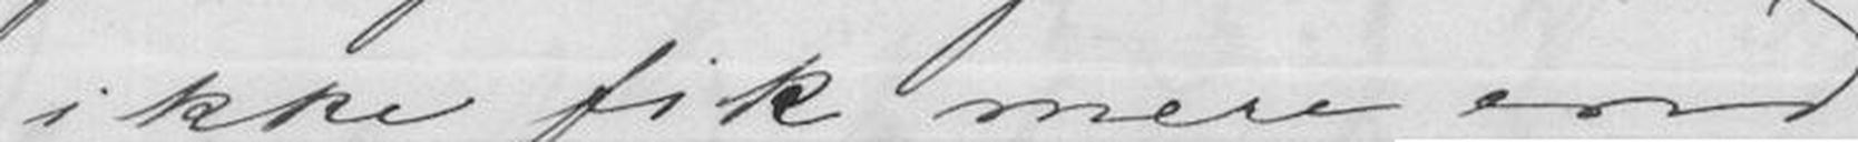ikke fik mere end
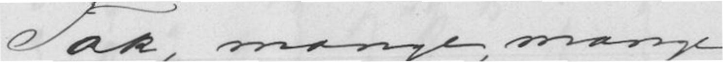Tak, mange, mange
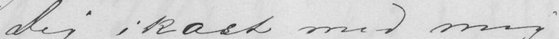Dig i kast med mig,
